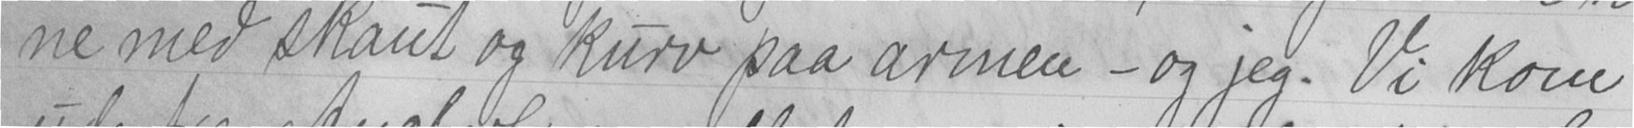ne med skaut og kurv paa armen - og jeg. Vi kom
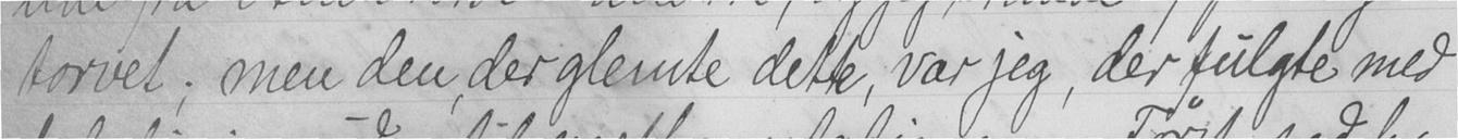torvet; men den der glemte dette, var jeg, der fulgte med
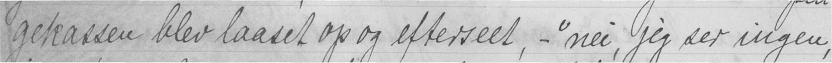gekassen blev laaset op og efterseet, - "nei, jeg ser ingen,
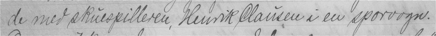de med skuespilleren, Henrik Clausen i en sporvogn.
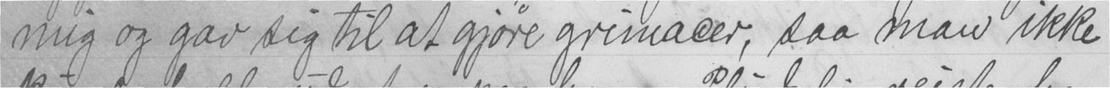mig og gav sig til at gjøre grimacer, saa man ikke
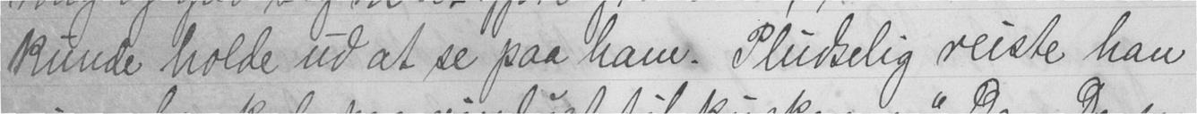kunde holde ud at se paa ham. Pludselig reiste han
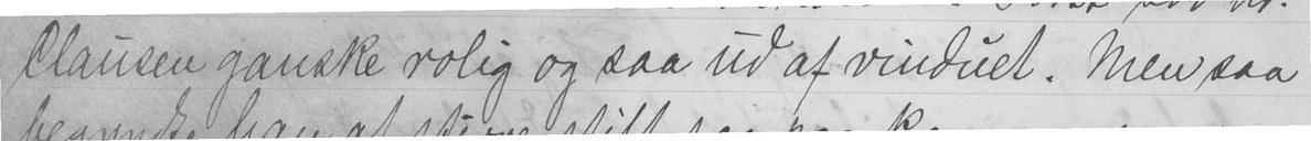Clausen ganske rolig og saa ud af vinduet. Men saa
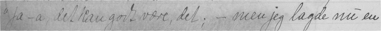"Ja-a, det kan godt være, det; - men jeg lagde nu en
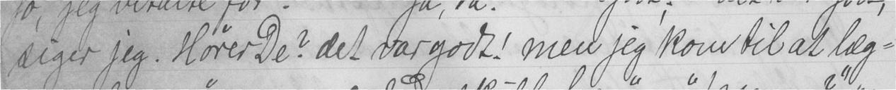siger jeg. Hører De? det var godt! men jeg kom til at læg-
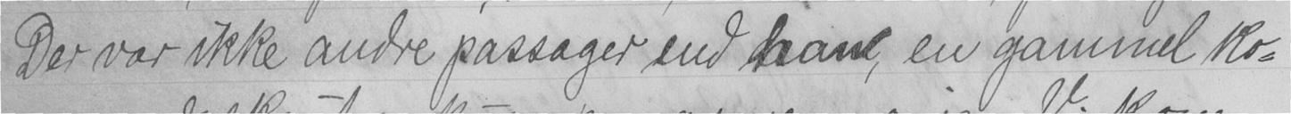Der var ikke andre passager end ham, en gammel ko-
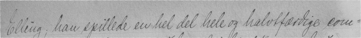Elling; han spillede en hel del hele og halvtfærdige com-
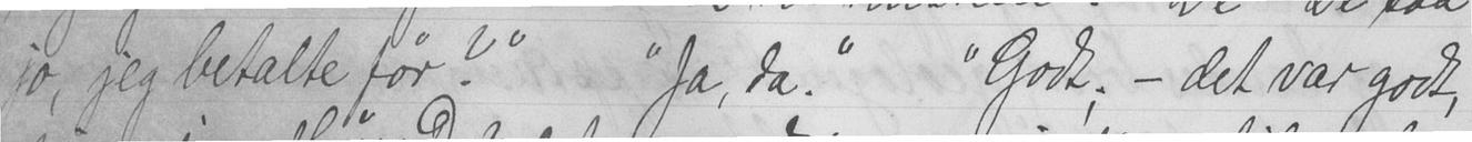jo, jeg betalte før?" "Ja, da." "Godt; - det var godt,
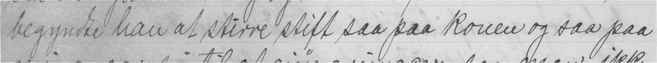begyndte han at stirre stift saa paa konen og saa paa
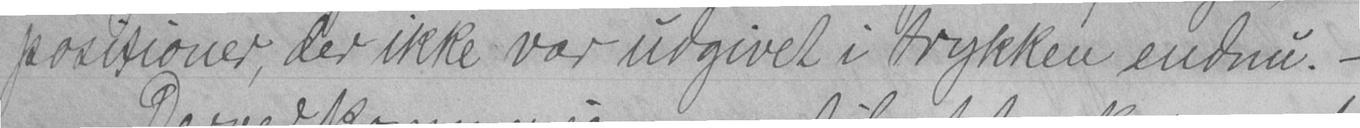positioner, der ikke var udgivet i trykken endnu. -
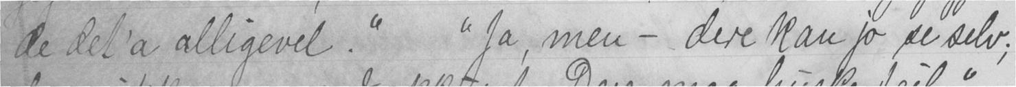de det'a alligevel." "Ja, men - dere kan jo se selv;
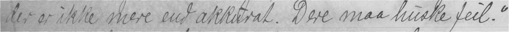der er ikke mere end akkurat. Dere maa huske feil."
